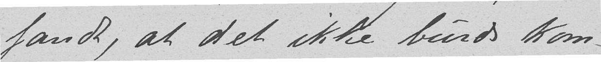fandt, at det ikke burde kom-
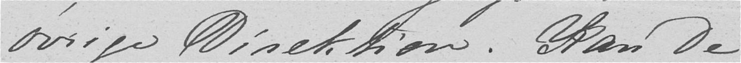øvige Direktion. Kan De
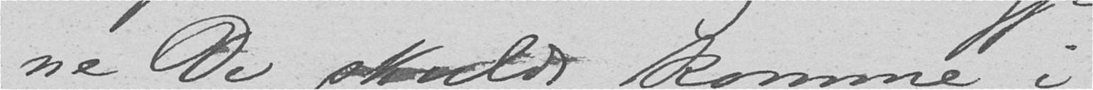ne De skulde komme i
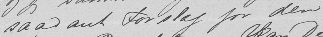saadant Forslag for den
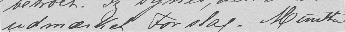udmærket Forslag. Munthe
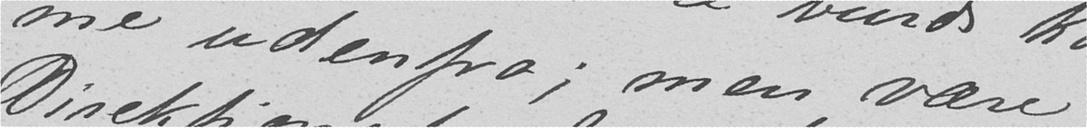me udenfra; men være
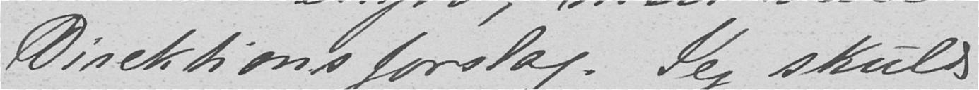Direktionsforslag. Jeg skulde
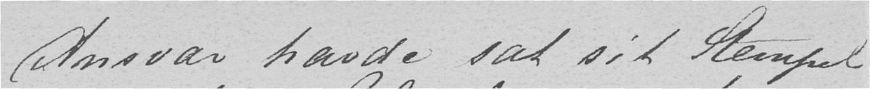Ansvar havde sat sit Stempel
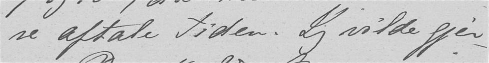re aftale Tiden. Jeg vilde gjer-
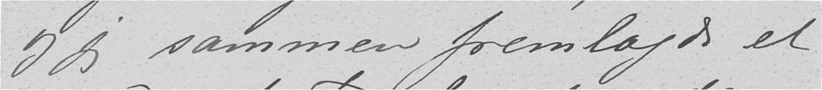og jeg sammen fremlagde et
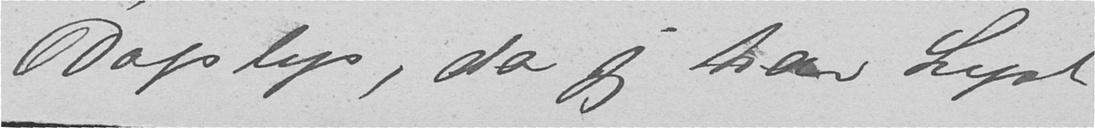Dagslys, da jeg har Lyst
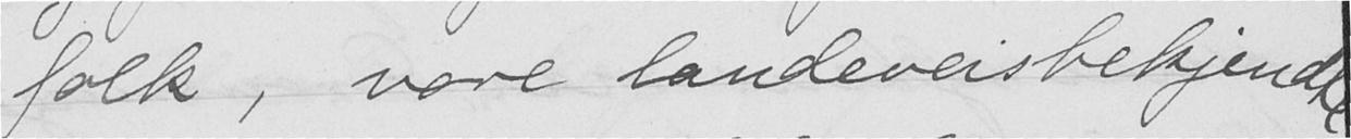folk, vore landeveisbekjendte
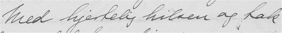Med hjertelig hilsen og tak
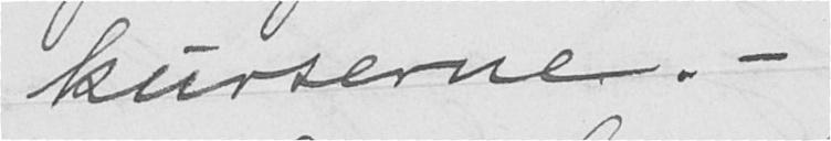kurserne. -
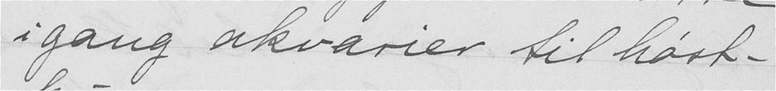i gang akvarier til høst-
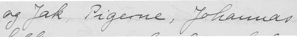og Jak, Pigerne, Johannas
